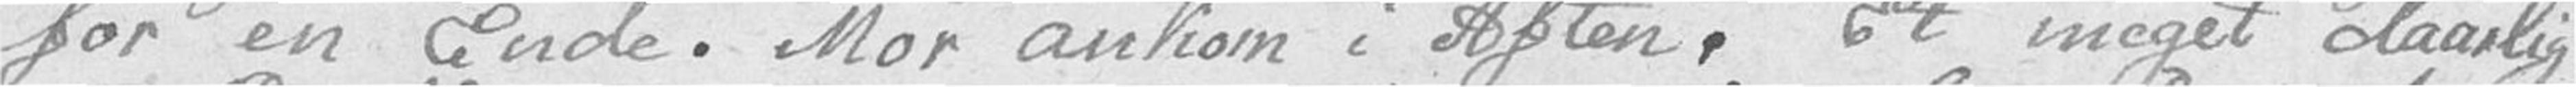for en Ende. Mor ankom i Aften. Et meget daarlig
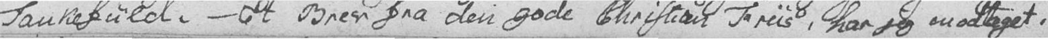Tankefuld. – Et Brev fra den gode Christian Friis, har jeg modtaget.
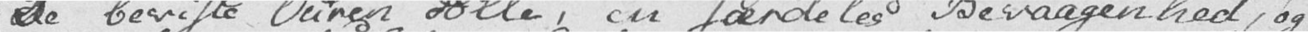De beviste Ouren Alle, en særdeles Bevaagenhed, og
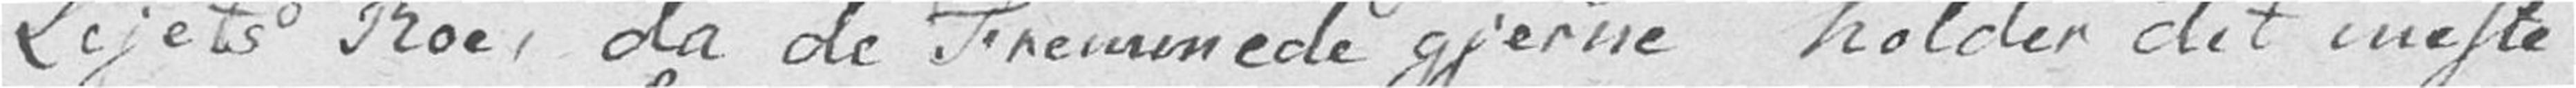Livets Roe, da de Fremmede gjerne holder det meste
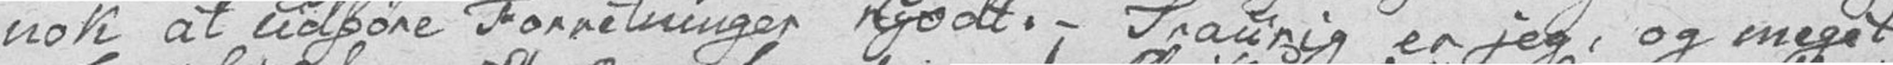nok at udføre Forretninger Godt. – Traurig er jeg, og meget
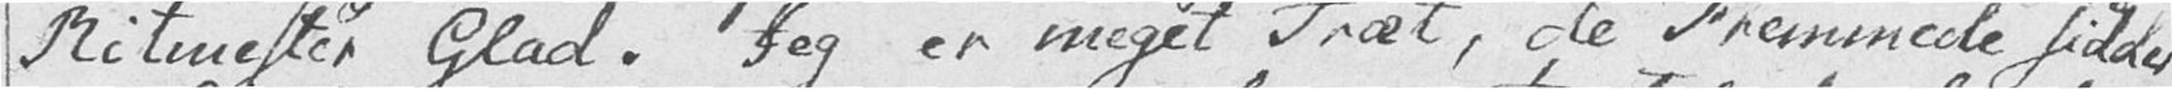Ritmester Glad. Jeg er meget Træt, de Fremmede sidder
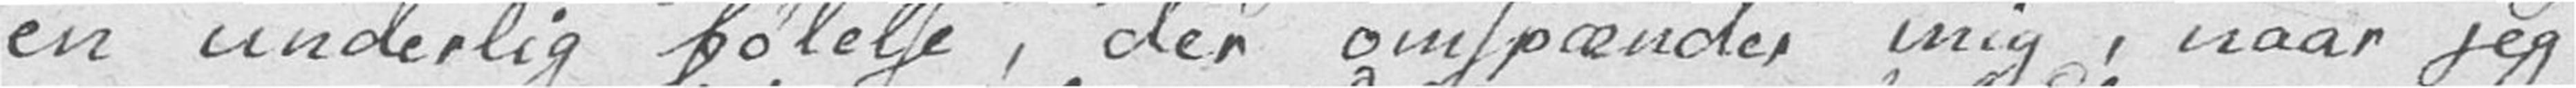en underlig følelse, der omspænder mig, naar jeg
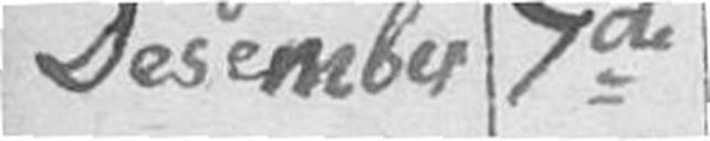Desember 7de
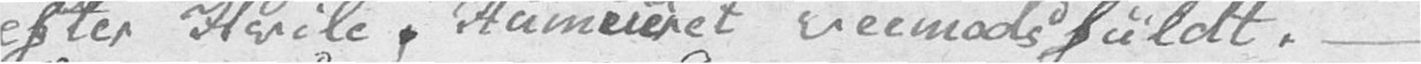efter Hvile. Humeuret veemodsfuldt. –
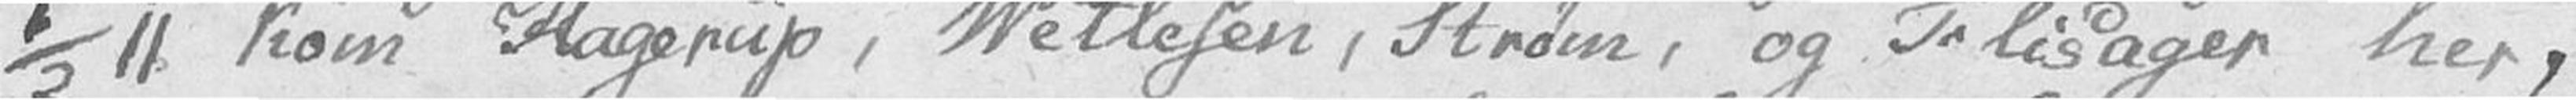½ 11 kom Hagerup, Vetlesen, Strøm, og Flisager her,
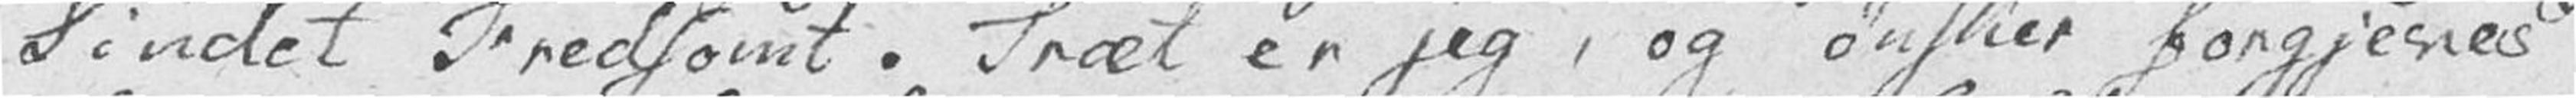Sindet Fredsomt. Træt er jeg, og ønsker forgjeves
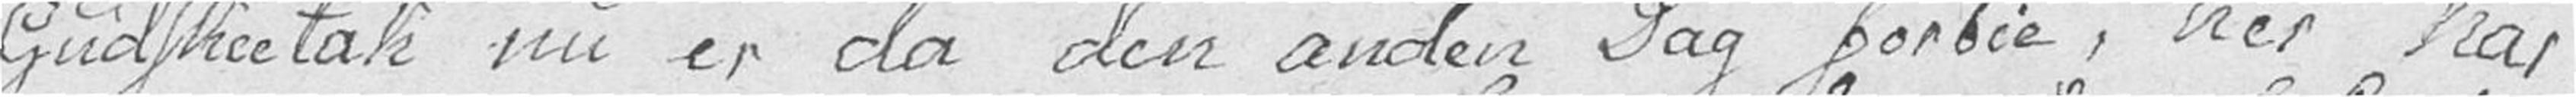Gudskeetak nu er da den anden Dag forbie, her har
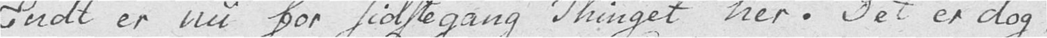Endt er nu for sidstegang Thinget her. Det er dog,
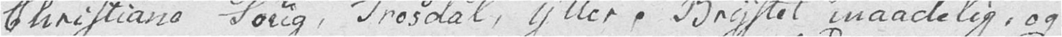Christian Soug, Trosdal, Ytter. Brystet maadelig, og
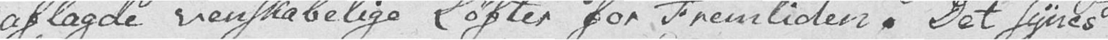aflagde venskabelige Løfter for Fremtiden. Det synes
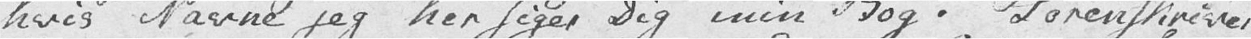hvis Navne jeg her siger Dig min Bog. Sorenskriver
